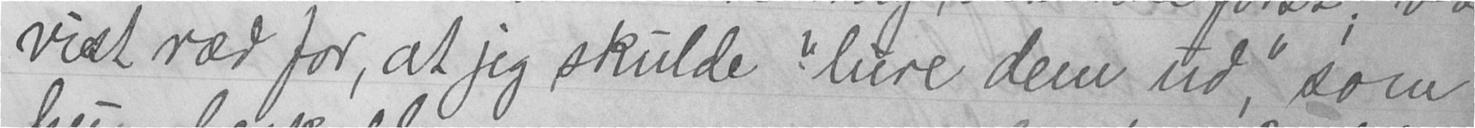vist ræd for, at jeg skulde "lure dem ud," som
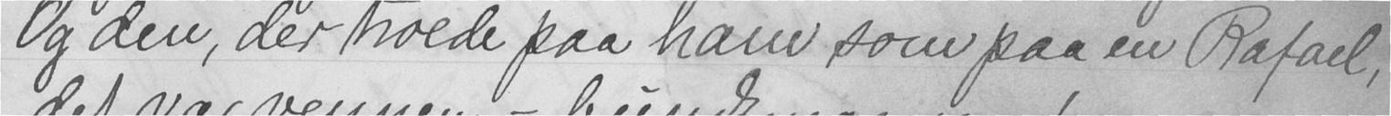Og den, der troede paa ham som paa en Rafael,
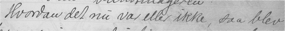Hvordan det nu var eller ikke, saa blev
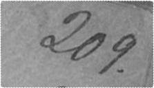209.
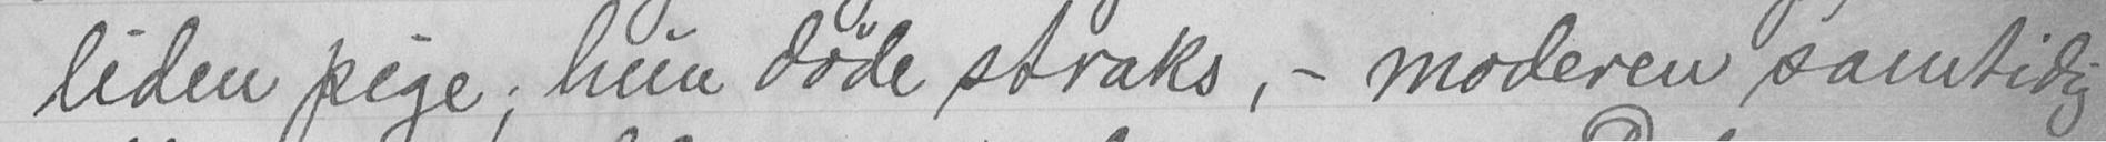liden pige; hun døde straks, - moderen samtidig
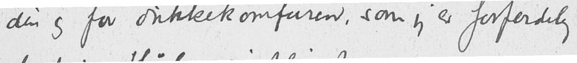din og for dukkekomfuren, som jeg er forferdelig
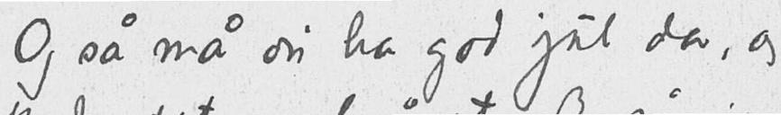Og sa må du ha god jul da, og
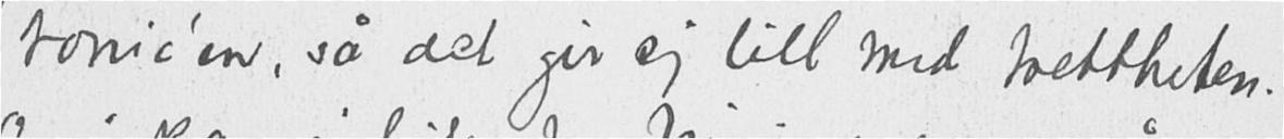tonic'en, så det gir sig litt med frettheten.
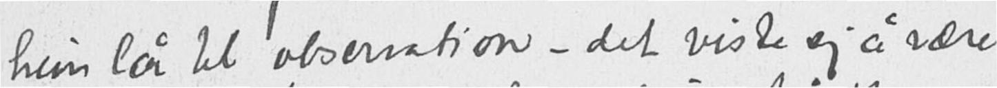hun lå tll observation – det viste sig å være
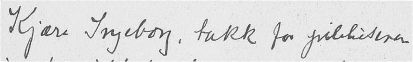Kjære Ingeborg, takk for julehilsenen
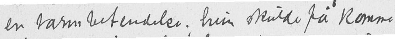en tarmbetendelse, hun skulde få komme
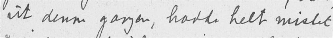ut denne gangen, hadde helt mistet
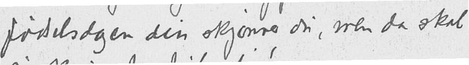fødselsdagen din skjønner du, men da skal
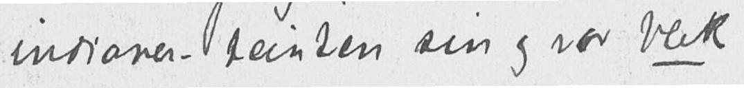indianer-teinten sin og var blek.
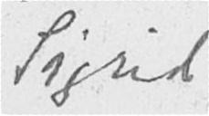Sigrid.
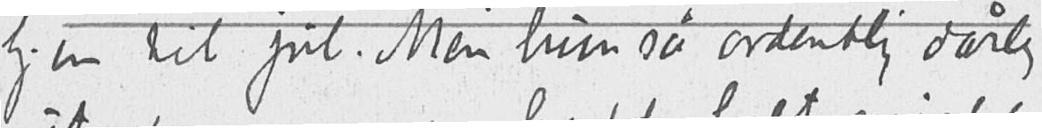hjem til jul. Men hun så ordentlig dårlig
